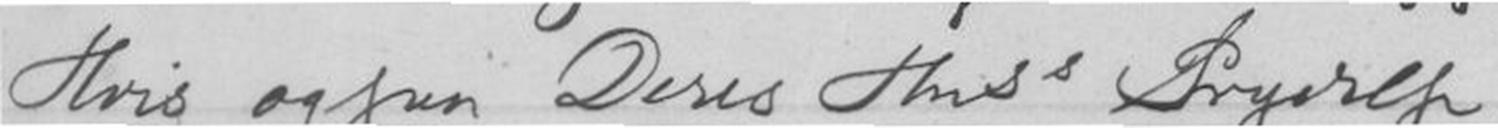Hvis ogsaa Deres Huss Prydelse
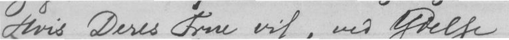Hvis Deres Frue vil, ved Ydelse
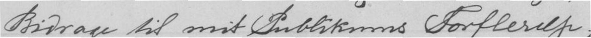Bidrage til mit Publikums Forflerelse,
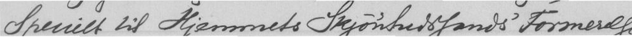Specielt til Hjemmets Skjønhedsstands Formerelse,
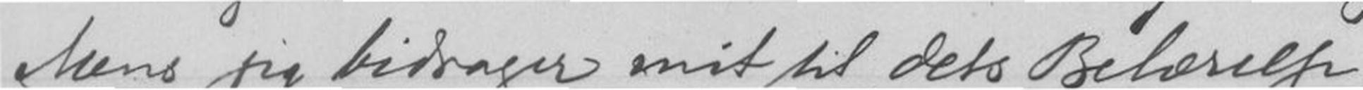Mens jeg bidrager mit til dets Belærelse,
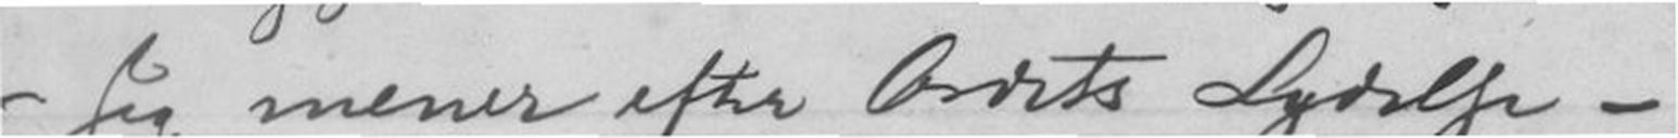- Jeg mener efter Orders Lydelse -
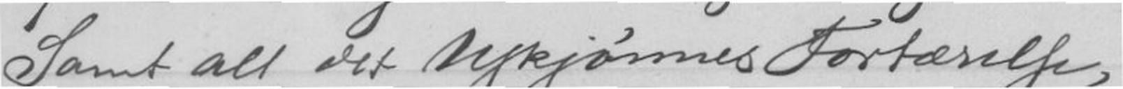Samt alt det Uskjønnes Fortærelse,
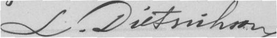L. Dietrichson
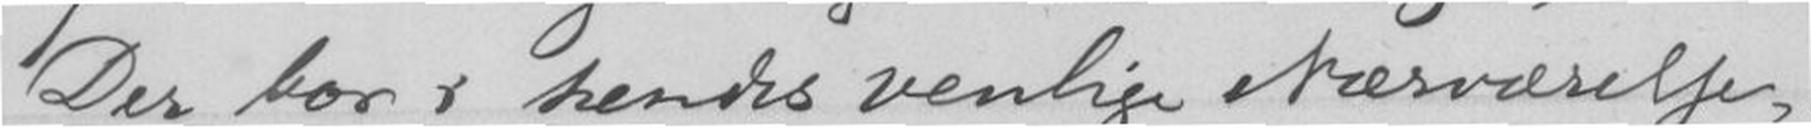Der har i hendes venlige Nærværelse,
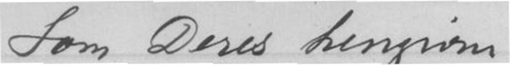Som Deres hengivne
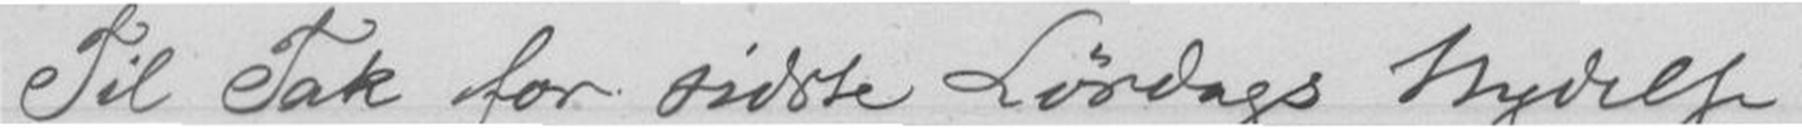Til Tak for sidste Lørdags Nydelse.
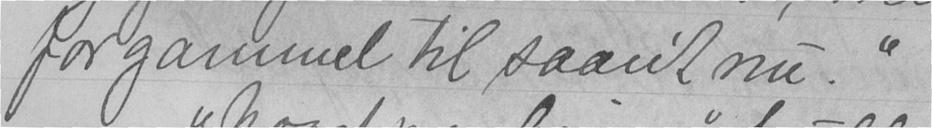for gammel til saan't nu. "
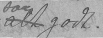alt godt.
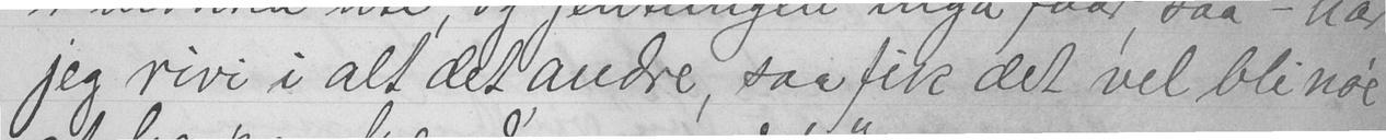jeg rivi i alt det andre, saa fik det vel bli no'e
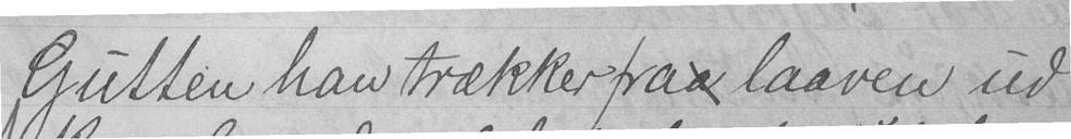Gutten han trækker fraa laaven ud
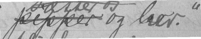pipper og ler."
